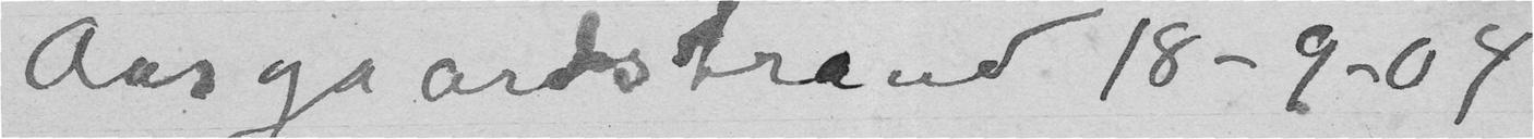Aasgaardstrand 18-9-04
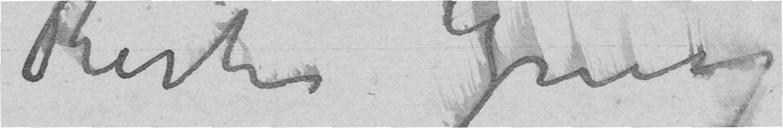Besten Grusz
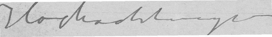Hochachtungsvoll
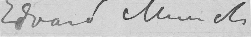Edvard Munch
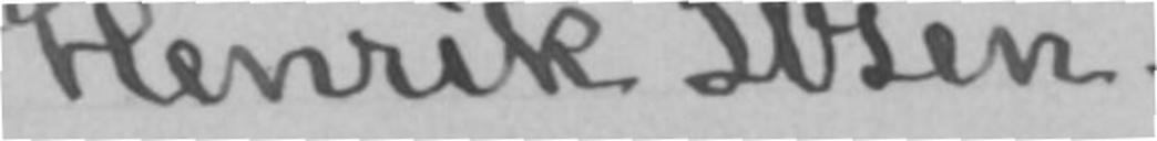Henrik Ibsen.
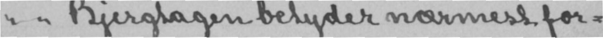" " Bjergtagen betyder nærmest for-
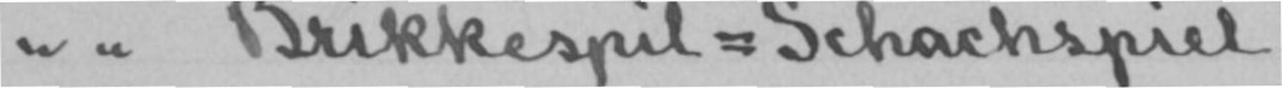" " Brikkespil = Schachspiel.
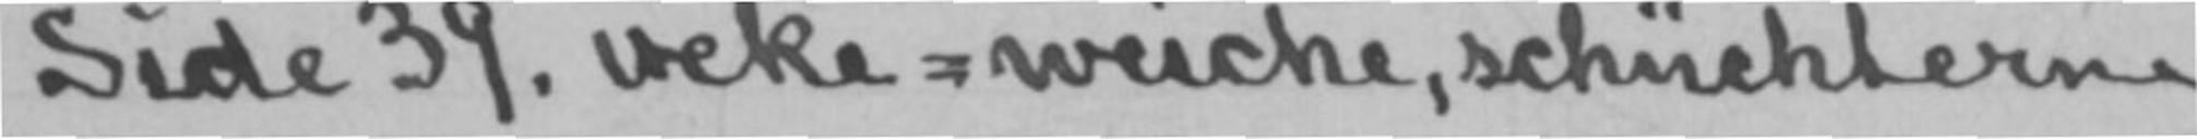Side 39. veke = weiche, schüchterne
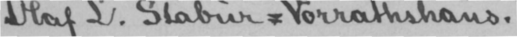Olaf L.Stabur = Vorrathshaus.
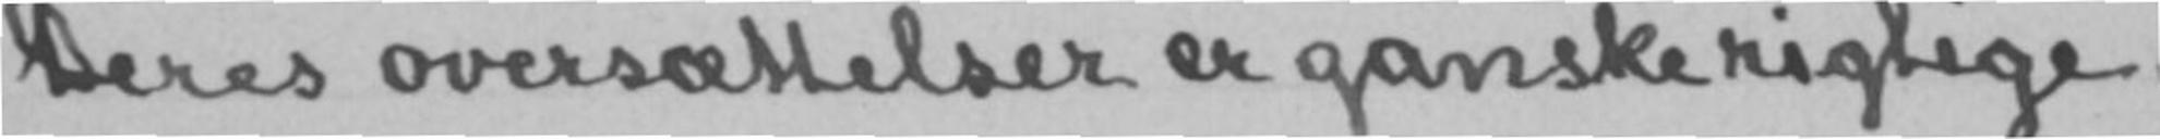Deres oversættelser er ganske rigtige.
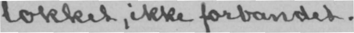lokket, ikke forbandet.
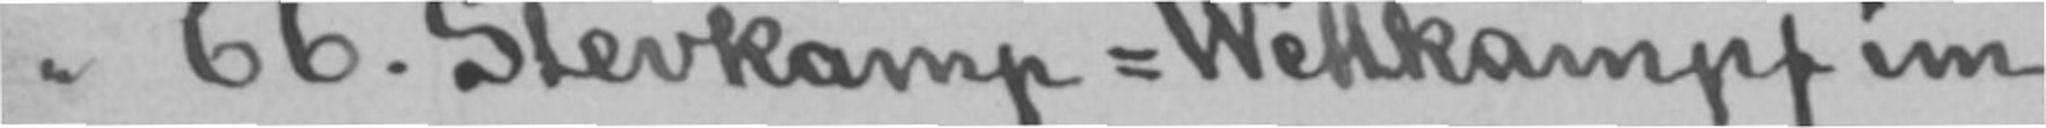" 66. Stevkamp = Wettkampf im
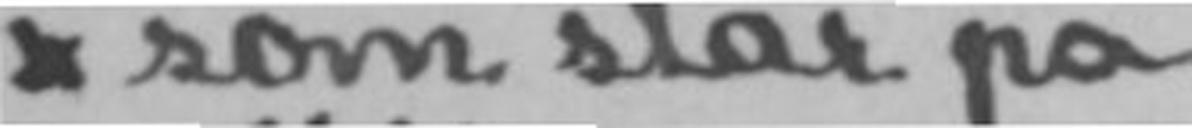* som står på
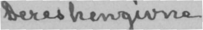Deres hengivne
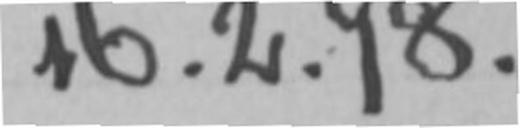16.2.98.
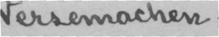Versemachen.
